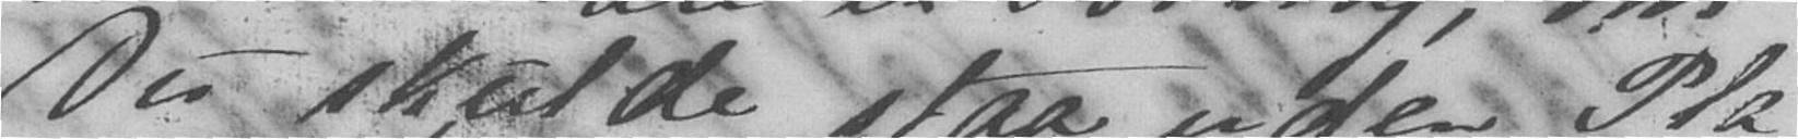Du skulde staa uden Pla-
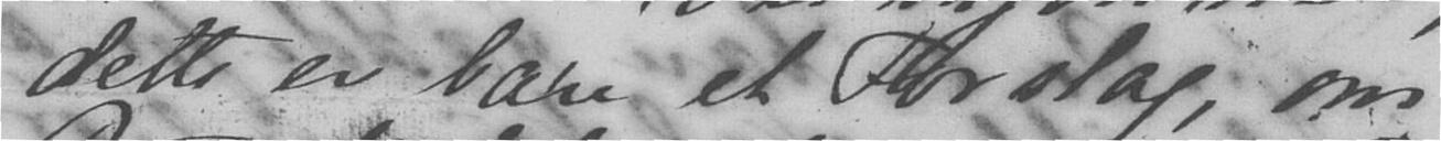dette er bare et Forslag, om
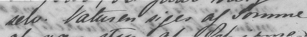selv. Naturen siges af Somme
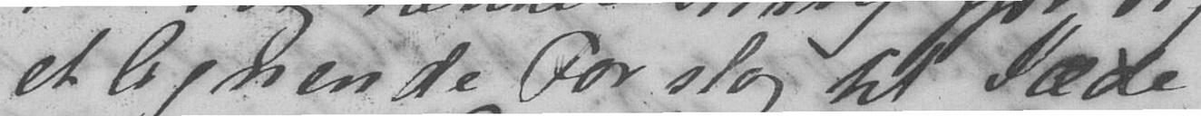et lignende Forslag til Sæde-
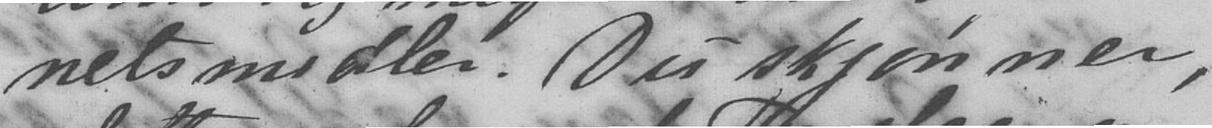netsmidler. Du skjønner,
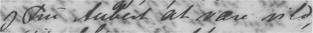og Fru Aubert at være vild,
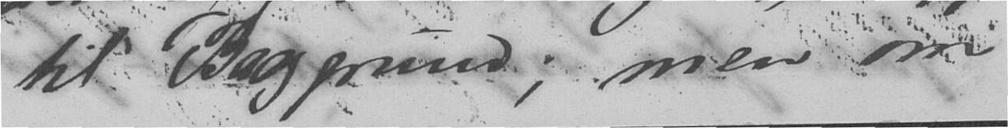til Baggrund; men om
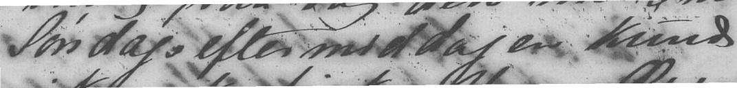Søndags eftermiddagen kunde
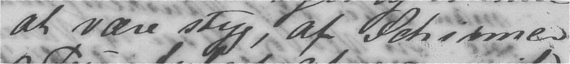at være styg, af Schirmer
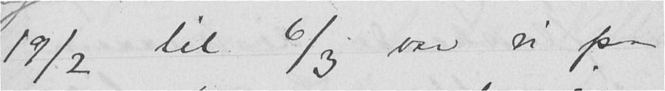19/2 til 6/3 var vi paa
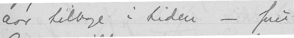aar tilbage i tiden - fru
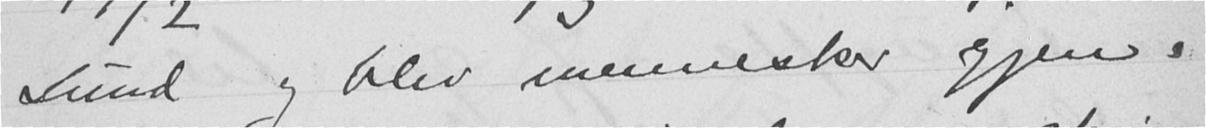Sund og blev mennesker igjen.
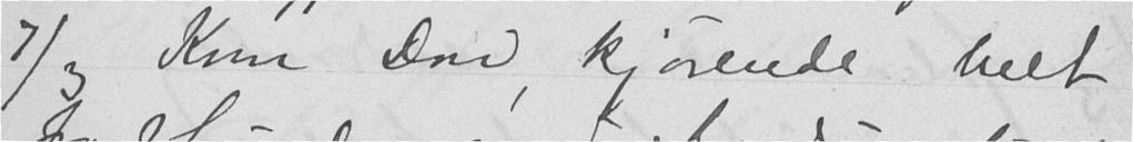7/3 Kom Don kjørende helt
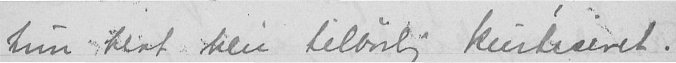hun blot blir tilbørlig kurtiseret.
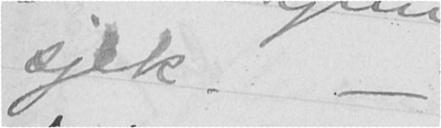sjek. -
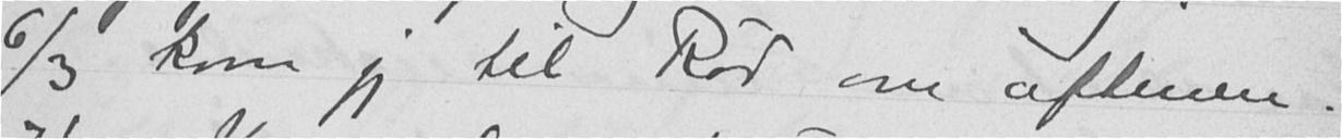6/3 kom jeg til Rød om aftenen.
